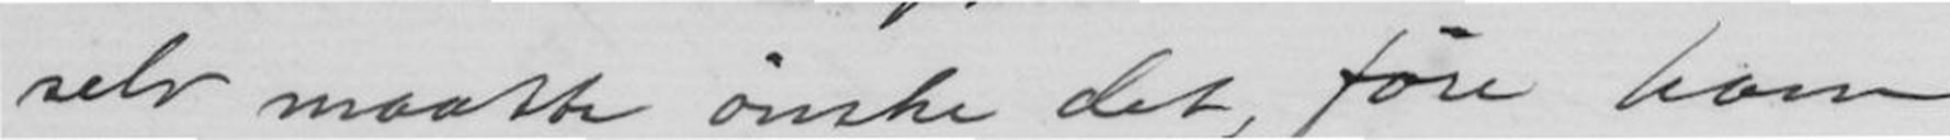selv maatte ønske det, føre ham
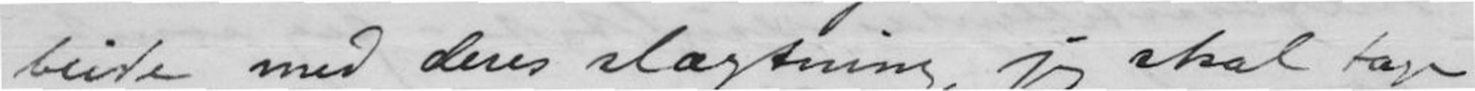beide med deres slægtning, jeg skal
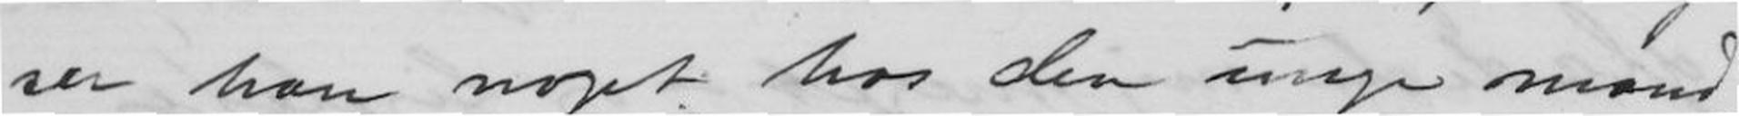ser han noget hos den unge mand,
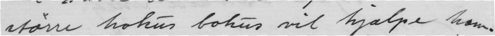større hokus bokus vil hjælpe ham.
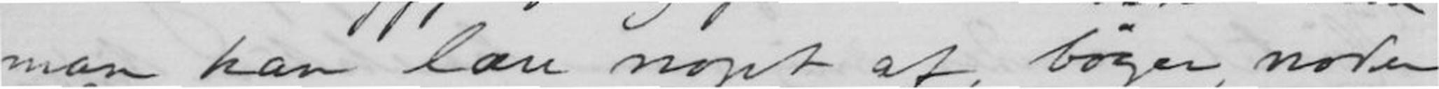man kan lære noget af, bøger, noder
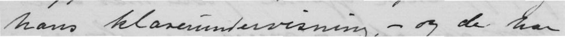hans klaverundervisning, - og de har
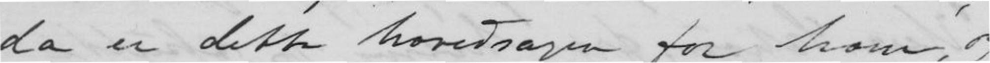da er dette hovedsagen for ham, og
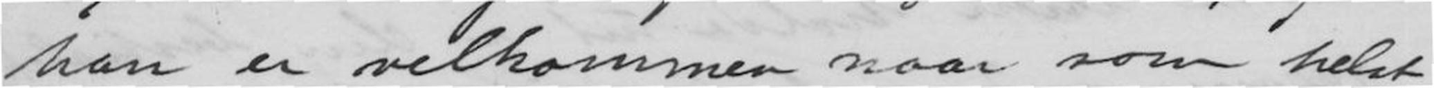han er velkommen naar som helst
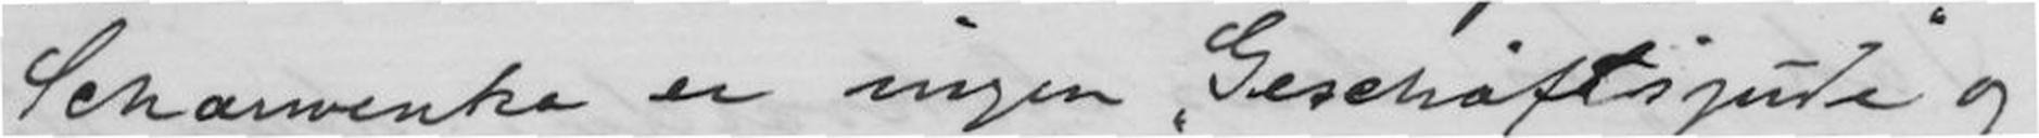Scharwenka er ingen Geschäftsgude og
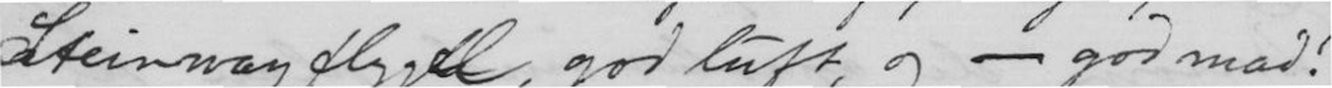Steinwayflygel, god luft, og - god mad!
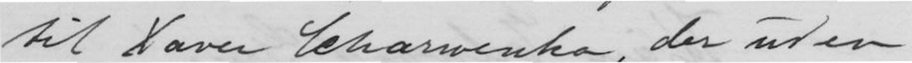til Xaver Scharwenka, der uden
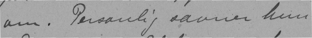om. Personlig savner hun
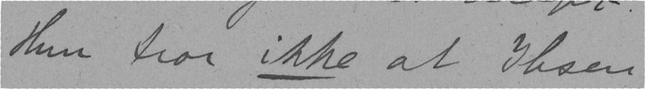Hun tror ikke at Ibsen
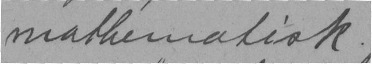mathematisk.
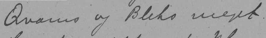Qvams og Blehs meget.
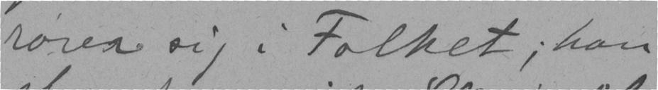rører sig i Folket; han
